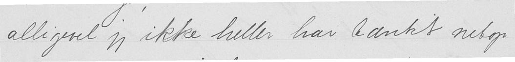alligevel jeg ikke heller har tænkt netop
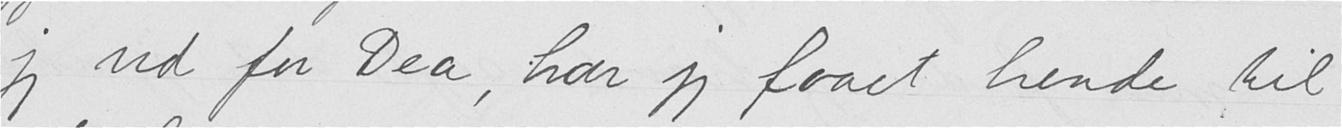jeg ud for Dea, har jeg faaet hende til
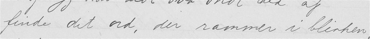finde det ord, der rammer i blinken,
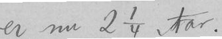er nu 2 1/4 stor.
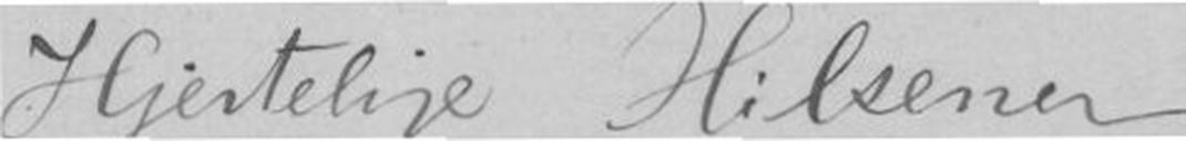Hjertelige Hilsener
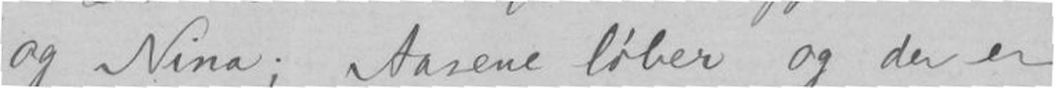og Nina; Aarene løber og der er
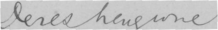Deres hengivne
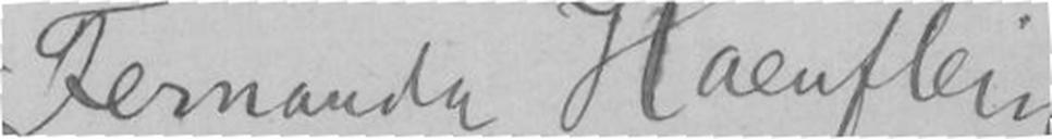Fernanda Haenflein.
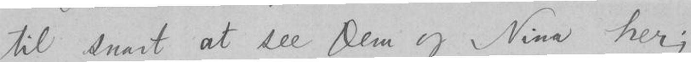til snart at see Dem og Nina her;
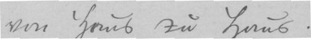"von Haus zu Haus".
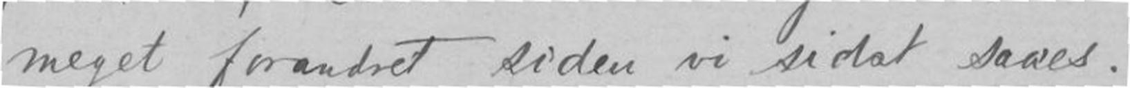meget forandret siden vi sidst saaes.
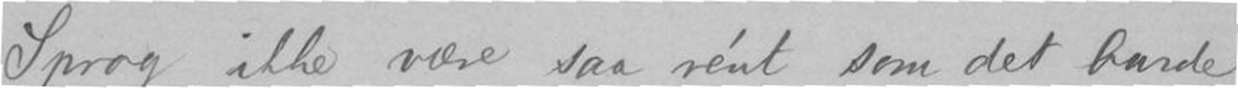Sprog ikke være saa rént som det burde
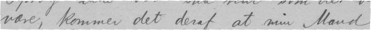være, kommer det deraf at min Mand
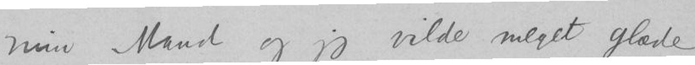min Mand og jeg vilde meget glæde
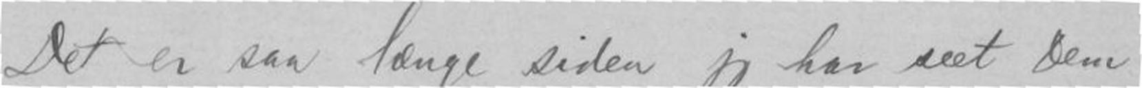Det er saa længe siden jeg har seet Dem
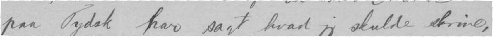paa Tydsk har sagt hvad jeg skulde skrive,
